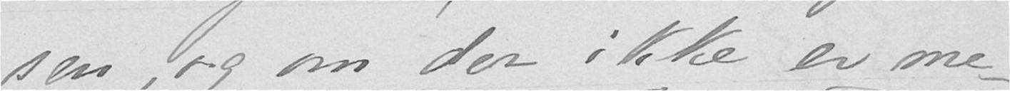sen, og om der ikke er me-
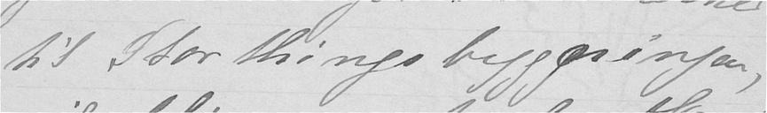til Storthingsbyggningen,
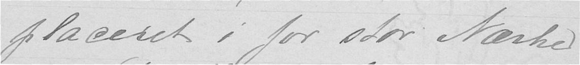placeret i for stor Nærhed
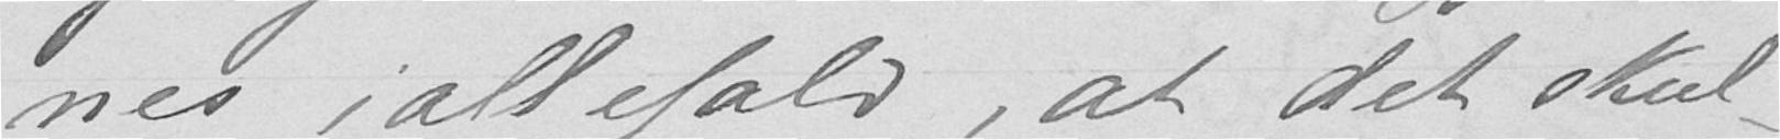nes iallefald, at det skul-
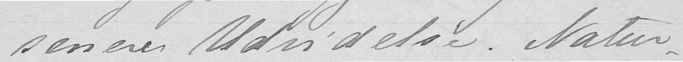senere Udvidelse. Natur-
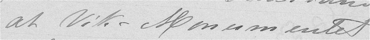at Vik-Monumentet
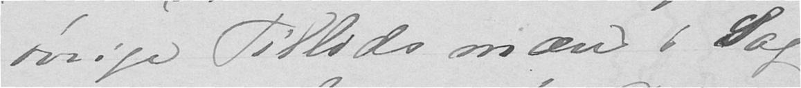øvrige Tillidsmænd i Sag-
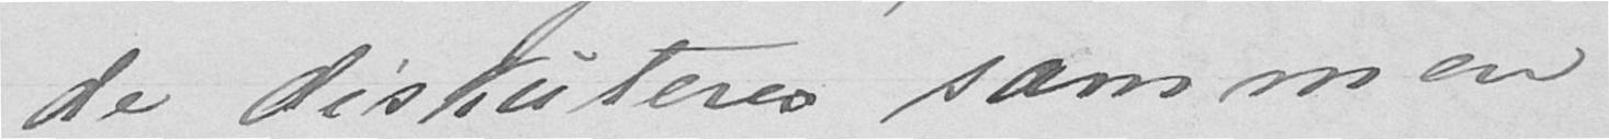de diskuteres sammen
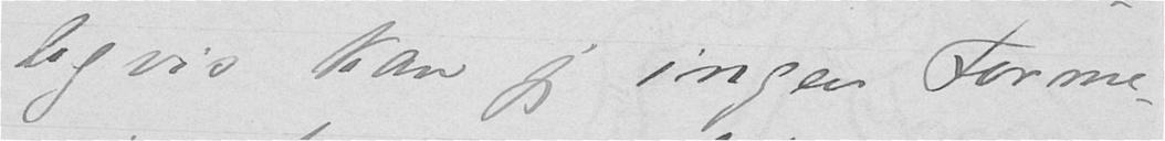ligvis kan jeg ingen Forme-
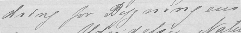dring for Bygningens
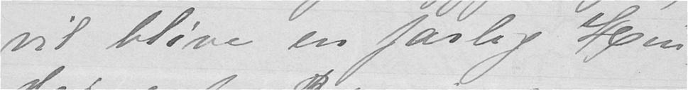vil blive en farlig Hin-
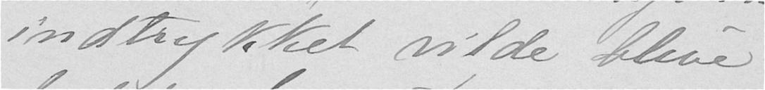indtrykket vilde blive
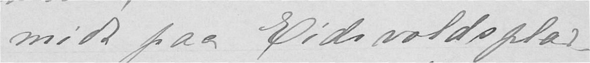midt paa Eidsvoldsplad-
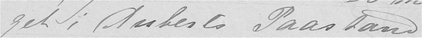get i Auberts Paastand
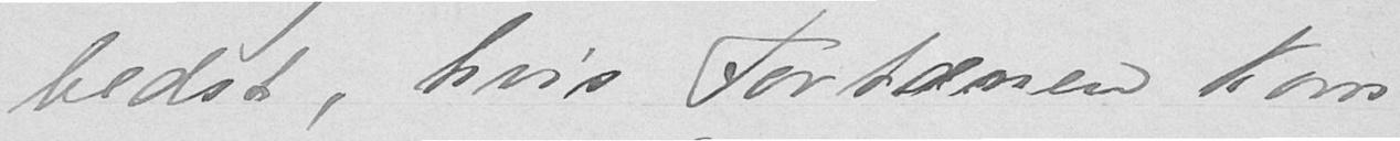bedst, hvis Fontænen kom
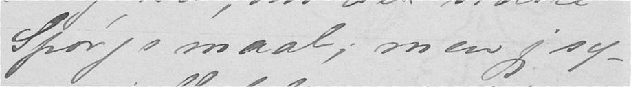Spørgsmaal; men jeg sy-
